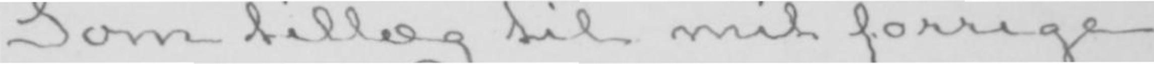Som tillæg til mit forrige
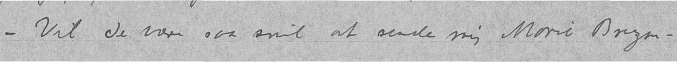- Vil De være saa snil at sende mig, Marie Bregen-
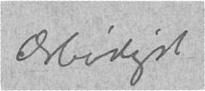Ærbødigst
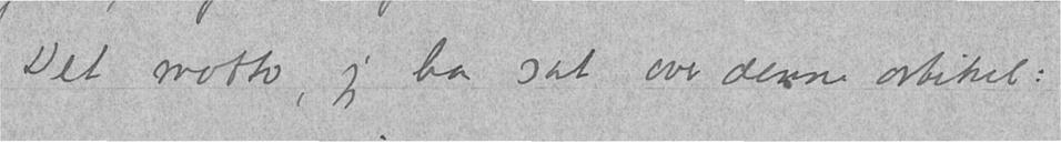Det motto, jeg har sat over denne artikel:
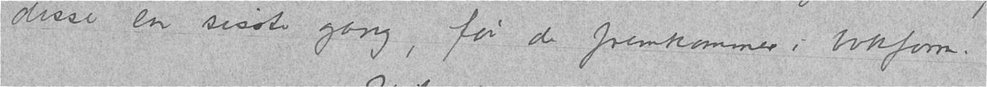disse en sisste gang, før de fremkommer i bokform.
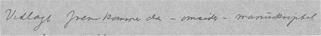Vedlagt fremkommer da – omsider – manuskriptet
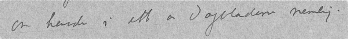om hende i ett av Dagbladene nemlig.
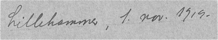Lillehammer, 1. nov. 1919.
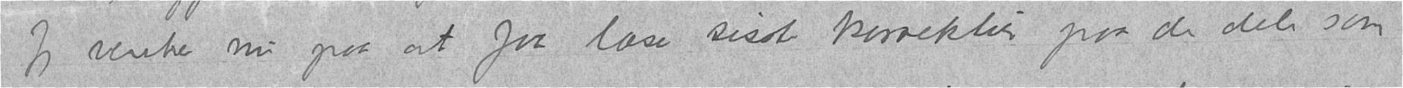Jeg venter nu paa at faa læse sisste korrektur paa de dele som
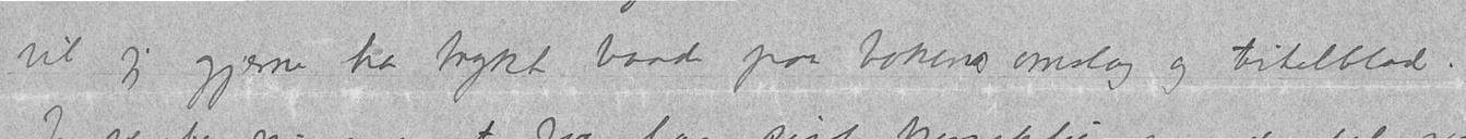vil jeg gjerne ha trykt baade paa bokens omslag og titelblad.
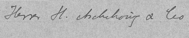Herrer H. Aschehoug & Co,
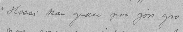"Hossi kan grase paa jori gro
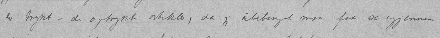er trykt – de optrykte artikler, da jeg ubetinget maa faa se igjennem
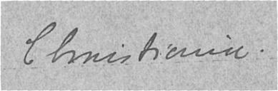Christiana.
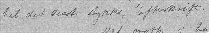til det sisste stykke, Efterskrift.
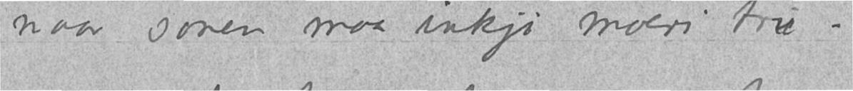naar sonen maa inkje moeri tru"
